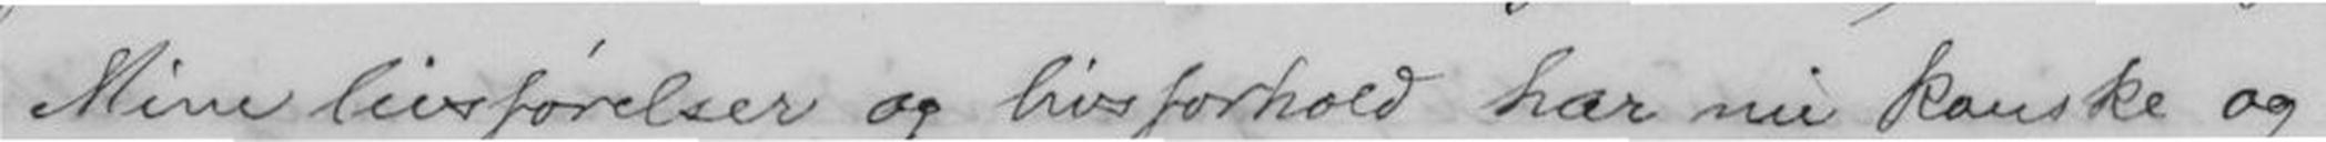Mine livsførelser og livsforhold har nu kanske og
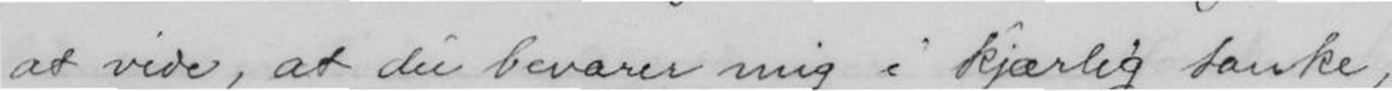at vide, at du bevarer mig i kjærlig tanke,
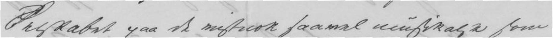Selskabet paa de vistnok saavel musikalske som
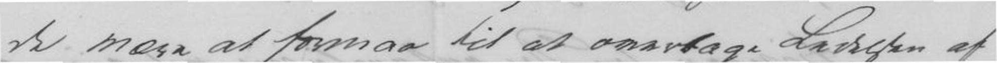de være at formaa til at overtage Ledelsen af
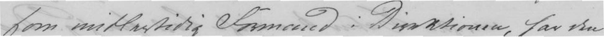som midlertidig Formand i Direktionen, har den
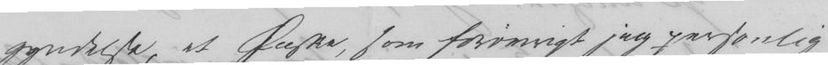gyndelse, et Ønske, som forøvrigt jeg personlig
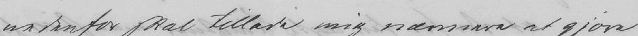nedenfor skal tillade mig nærmere at gjøre
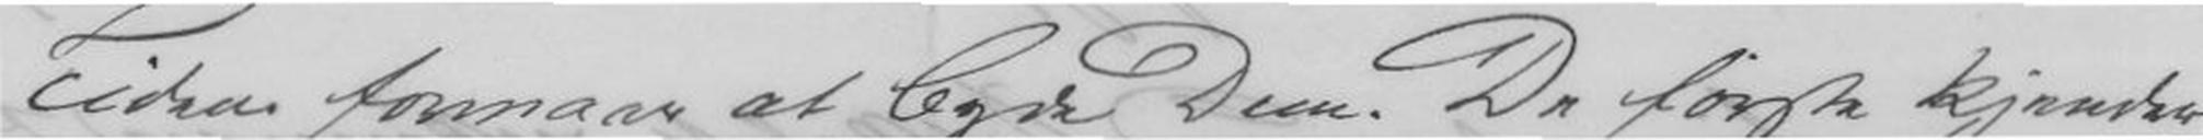Tiden formaar at byde Dem. De første kjender
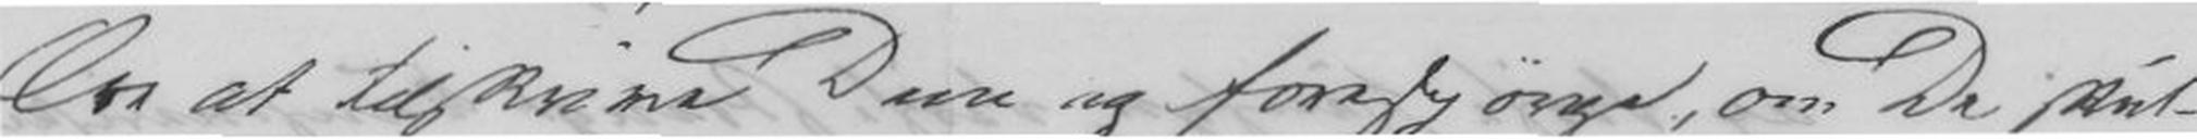Ære at tilskrive Dem og forespørge, om De skul-
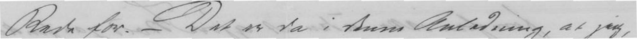Rede for. - Det er da i denne Anledning, at jeg,
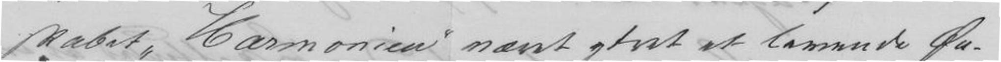skabet Harmonien været ytret et levnede Øn-
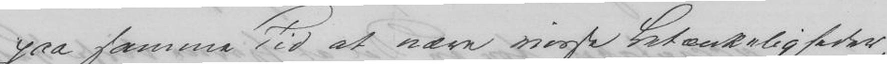paa samme Tid at nære visse Betænkeligheder,
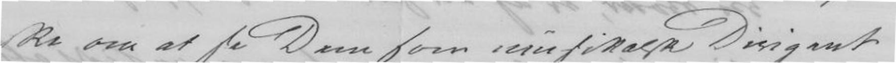ske om at se Dem som musikalsk Dirigent
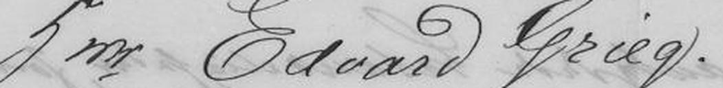Hrr Edvard Grieg.
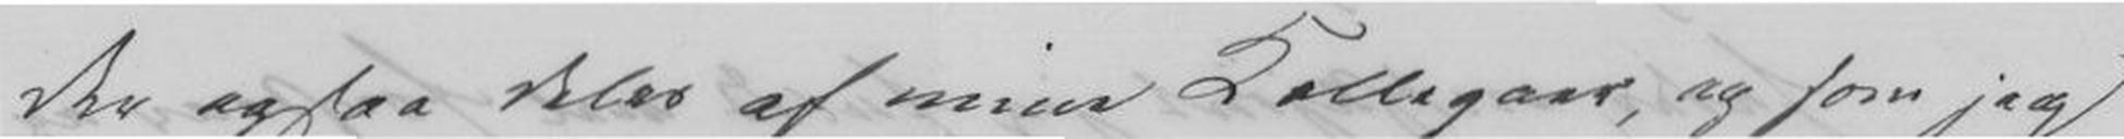der ogsaa deles af mine Kollegaer, og som jeg
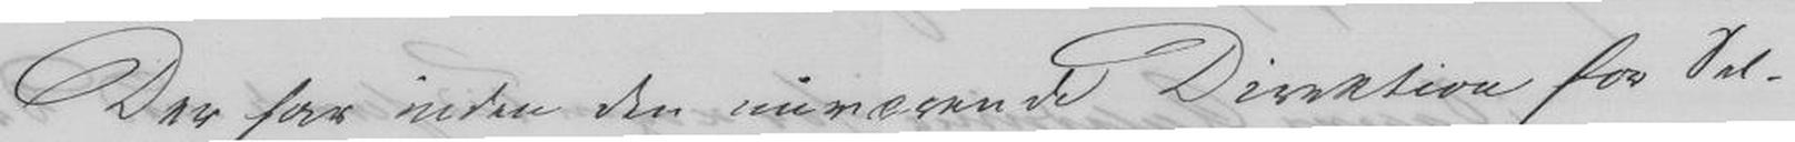Der have inden den nuværende DireKtion for Sel-
